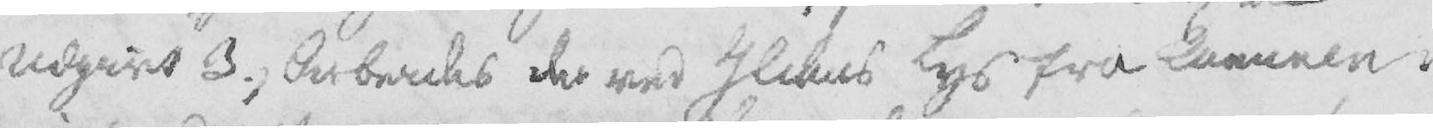Udgivt 3., Arbeides der ved Ildens Lys fra Caminen.
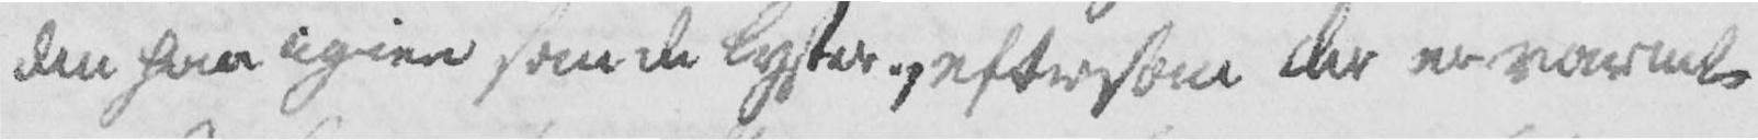den paa igien som de lyster, eftersom der er varmt
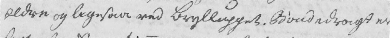ældre og ligesaa ved Brylluppet. Bondedragt er
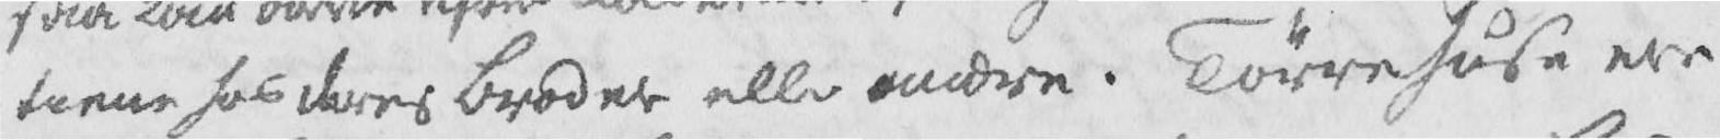tiene hos deres Broder alle andre. Tørrehuse ere
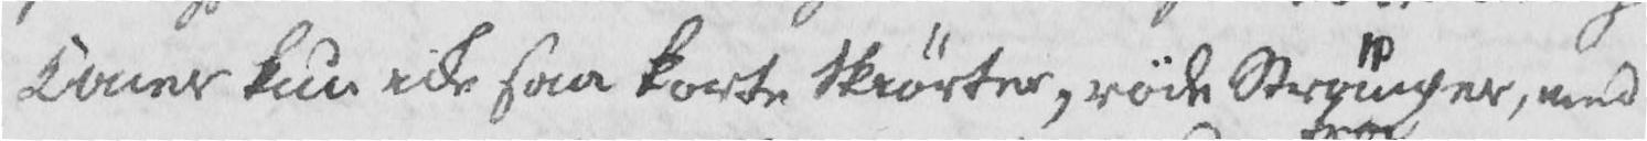Koner kun icke saa korte Skiørter, røde Strømper, med
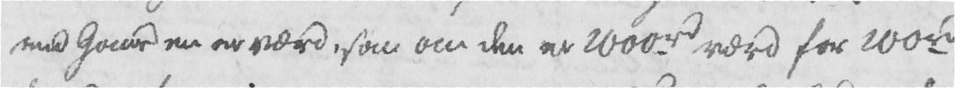end Gaarden er værd, som om den er 2000 rd værd for 200 rd
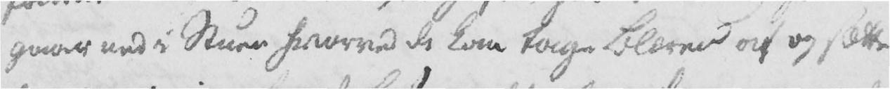gaar ned i Stuen hvorved de kan tage Blæren af og sætte
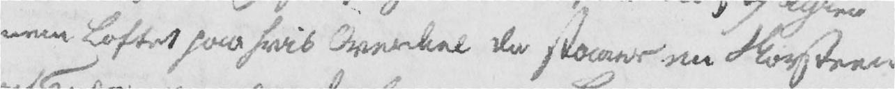nem Loftet paa hvis Overdel der staaer en Skorsteen
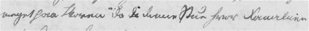meget paa Skoven da i denne Stue hvor Familien
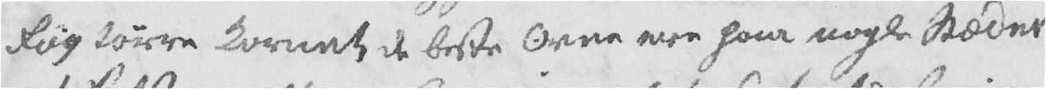Røg tørre Kornet de beste Ovne ere paa nogle Stæder
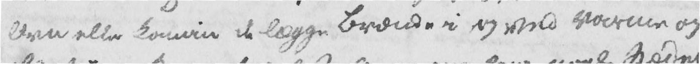Ovn eller Kamin de lægge Brænde i og ved Varme og
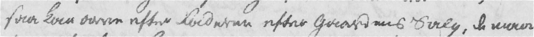saa kan arve efter Faderen efter Gaardens Salg, de maa
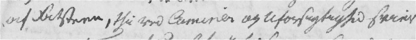af Fetsteen, thi ved Caminer og Uforsigtighed svier
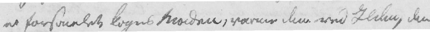er forsamlet koges Maden, varmer den ved Ilden, den
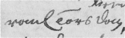vanl Torsdag,
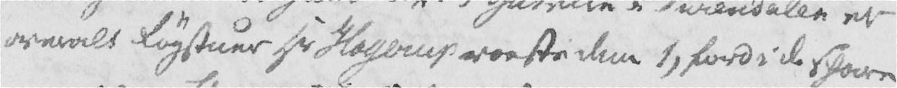overalt Røgstuer Hr Hagerup rooste dem 1, fordi de spare
